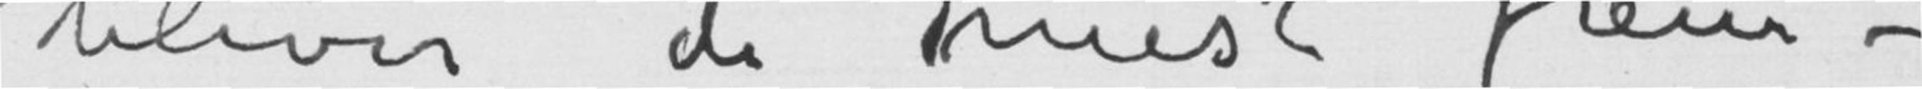bliver de mest frem-
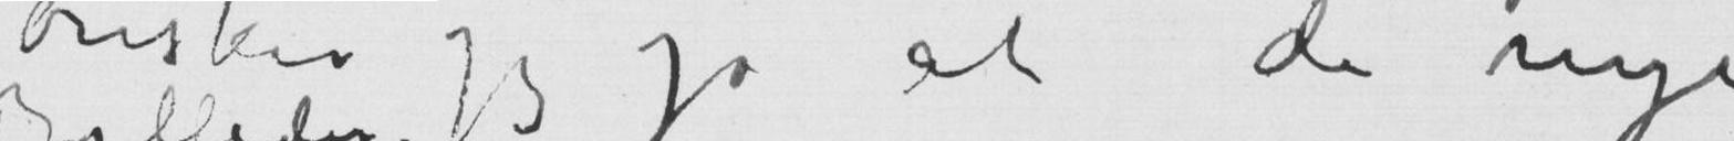ønsker jeg joat de nye
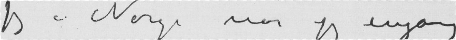jeg i Norge når jeg engang
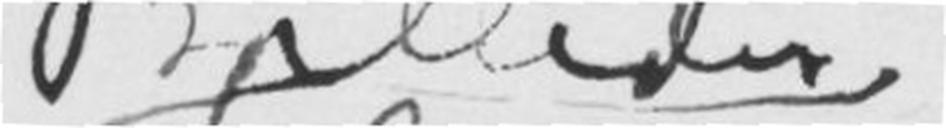Billeder
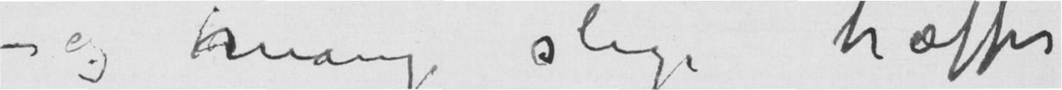– og mange slige træffer
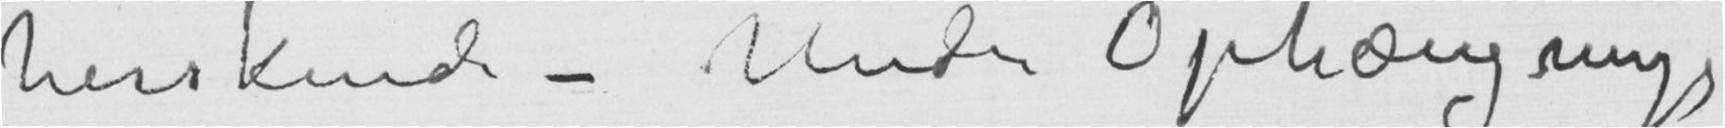herskende – Under ophængning
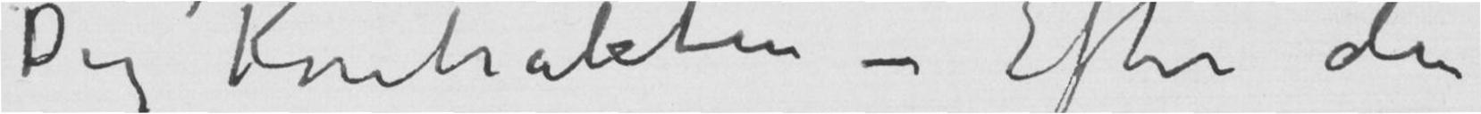Dig Kontrakten – Efter den
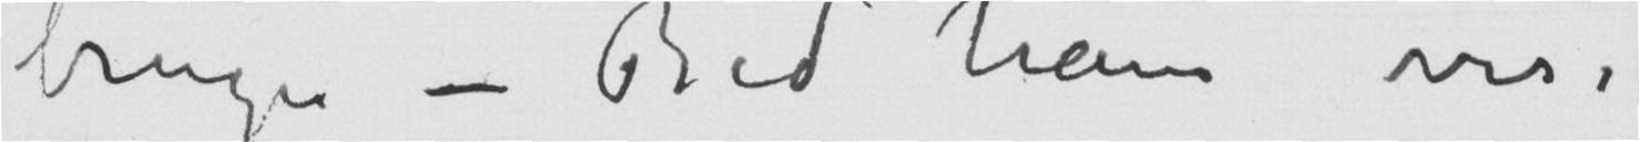bruges – Bed ham vise
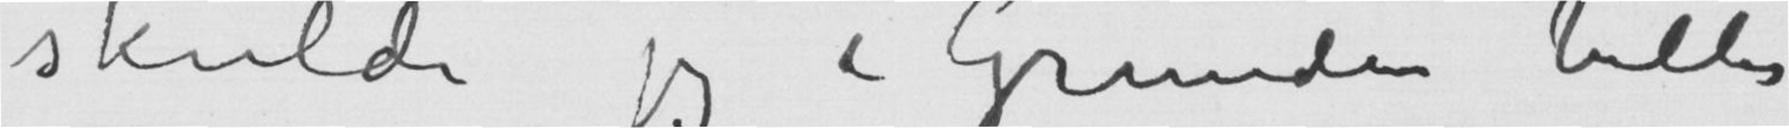skulde jeg i Grunden heller
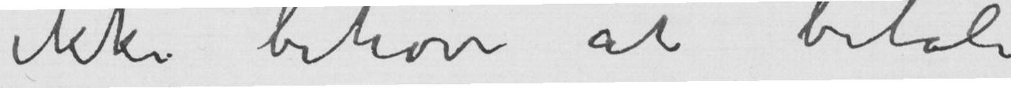ikke behøve at betale
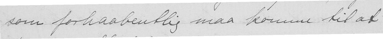som forhaabentlig maa komme til at
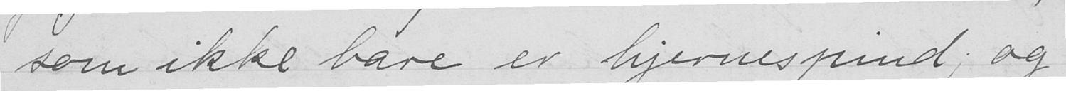som ikke bare er hjernespind; og
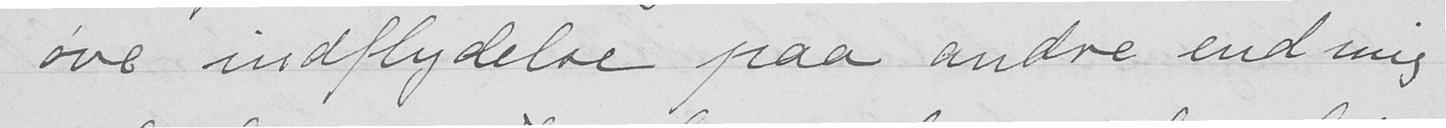øve indflydelse paa andre end mig
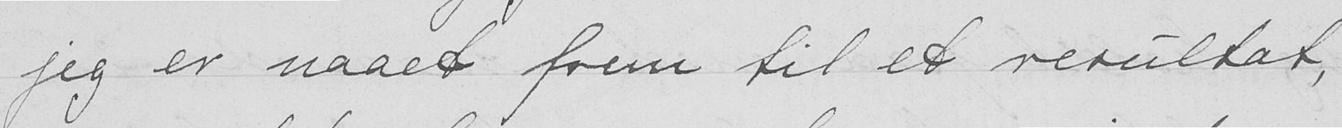jeg er naaet frem til et resultat,
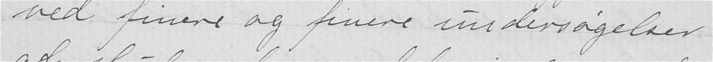ved finere og finere undersøgelser
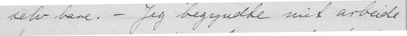selv bare. - Jeg begyndte mit arbeide
